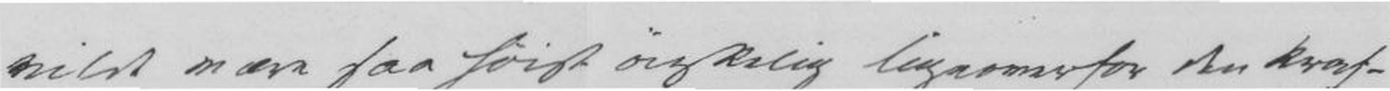vilde være saa høist ønskelig ligeoverfor den kraf-
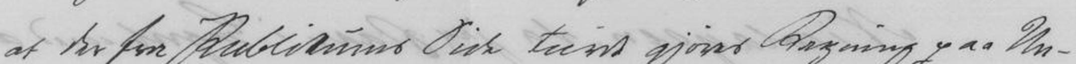at der fra Publikums Side turde gjøres Regning paa Un-
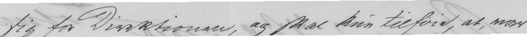sig for Direktionen, og skal kun tilføre, at være
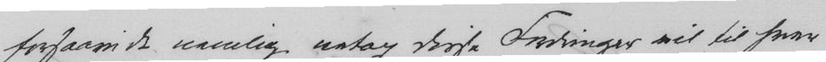forsaavidt nemlig netop disse Fordringer vil til svar
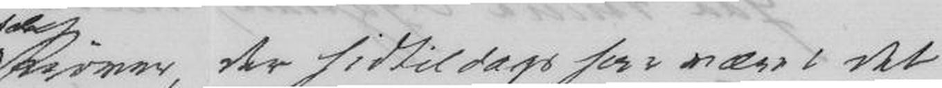Prøver, der hidtil dags have været i det
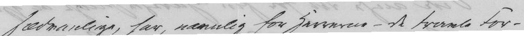sædvanlige, har, nemlige for Herrerne - de troende For-
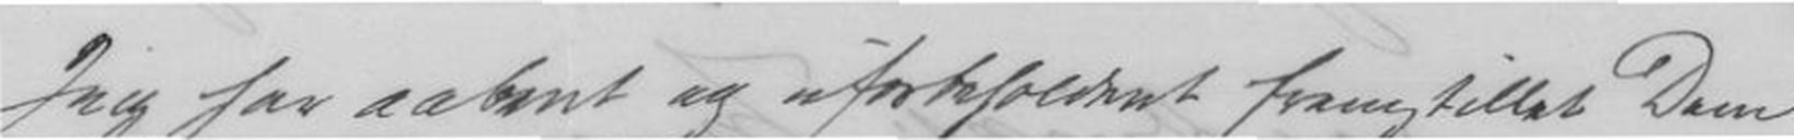Jeg har aabent og uforbeholdent fremstillet Dem
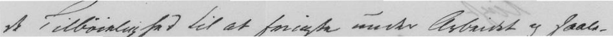de Tilbøielighed til at svigte under Arbeidet og haal-
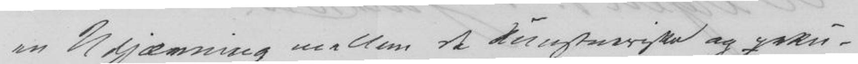en Udjævning mellem de kunstneriske og pekun-
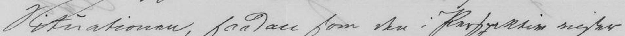Situationen, saadan som den i Prospektiv viser
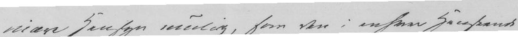niære Hensyn mulig, som den i enhver Henseende
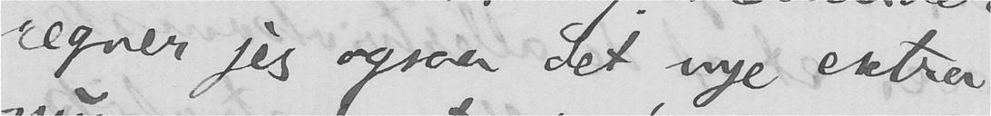regner jeg ogsaa det nye extra-
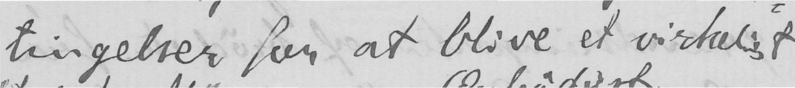tingelser for at blive et virkeligt
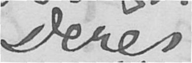Deres
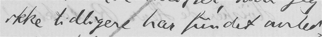ikke tidligere har fundet anled-
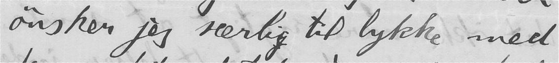ønsker jeg særlig til lykke med
